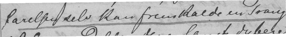farelser selv kan fremkalde en Trang
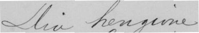Din hengivne
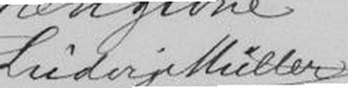Ludvig Müller
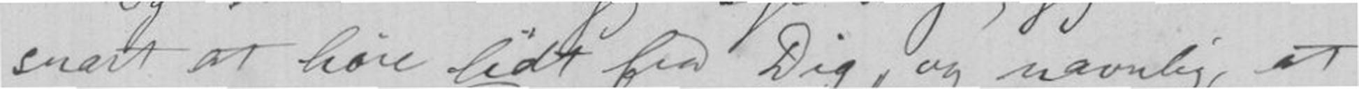snart at høre lidt fra Dig, og navnlig, at
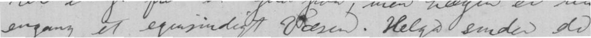engang et egensindigt Væsen. Helga sender de
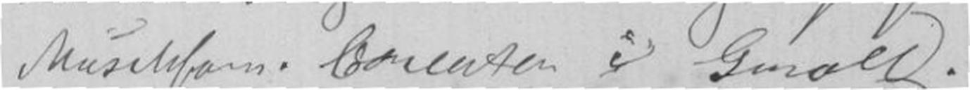Musikforn. Concerten i Gmoll.
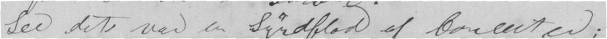See det var en Syndflod af Concerter;
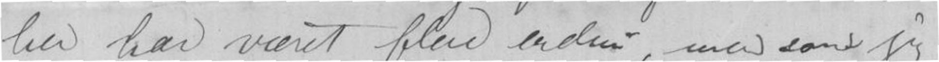her har været flere endnu, men som jeg
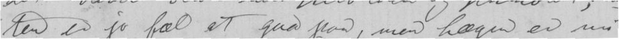ten er jo fæl at gaa paa, men Lægen er nu
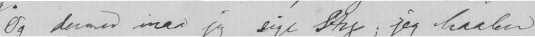Og dermed maa jeg sige Stop; jeg haaber
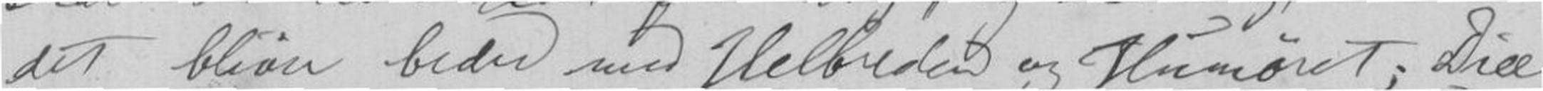det bliver bedre med Helbreden og Humøret; Diæ-
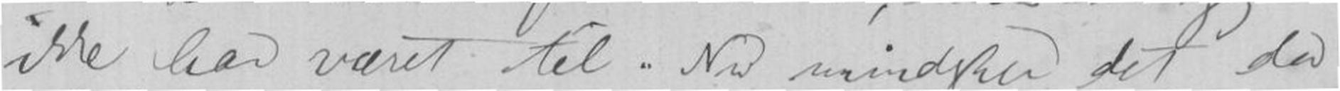ikke har været til. Nu mindsker det da
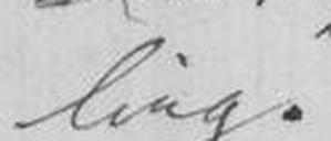ling.
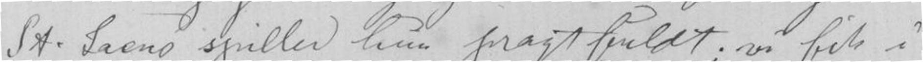St. Saens spiller hun pragtfuldt; vi fik i
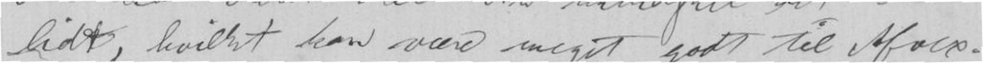lidt, hvilket kan være meget godt til Avex.-
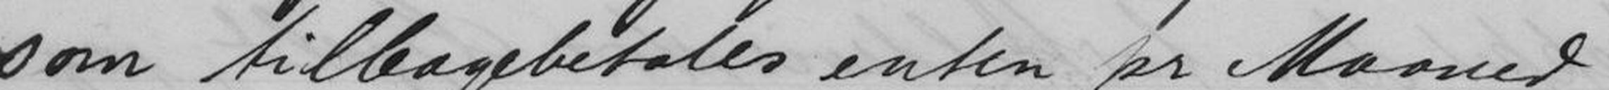som tilbagebetales enten pr Maaned
